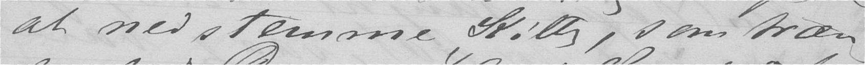at nedstemme Kitty, som træn-
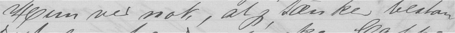Hun ved nok, at jeg tænker bestan-
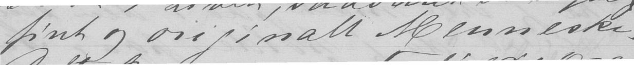fint og originalt Menneske.
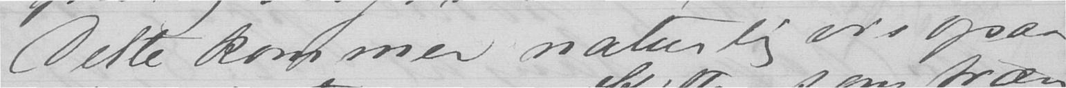Dette kommer naturligvis ogsaa
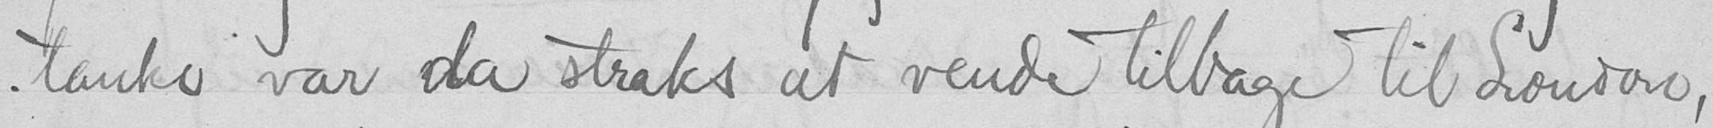tanke var da straks at vende tilbage til London,
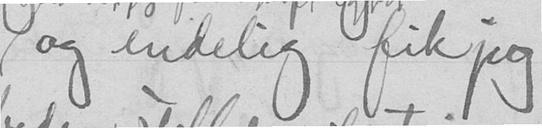og endelig fik jeg
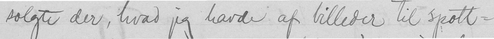solgte der, hvad jeg havde af billeder til spott-
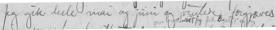Jeg gik hele mai og juni og ventede forgjæves
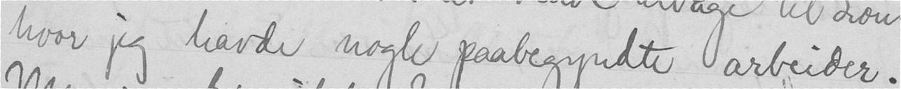hvor jeg havde nogle paabegyndte arbeider.
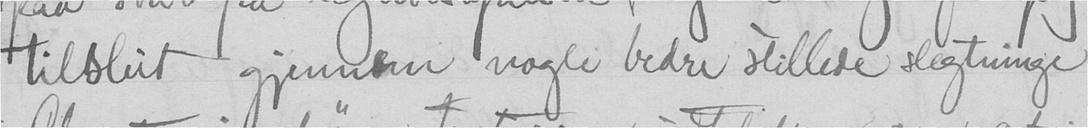tilslut gjennem nogle bedre stillede slegtninge
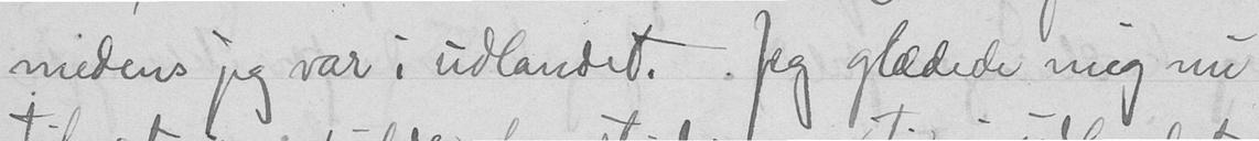medens jeg var i udlandet.. Jeg glædede mig nu
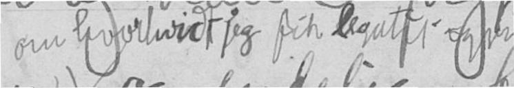om hvorhvidt jeg fik legatet igjen
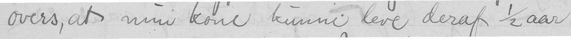overs at min kone kunne leve deraf 1/2aar
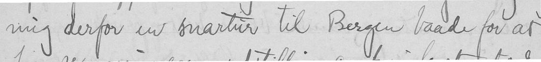mig derfor en snartur til Bergen baade for at
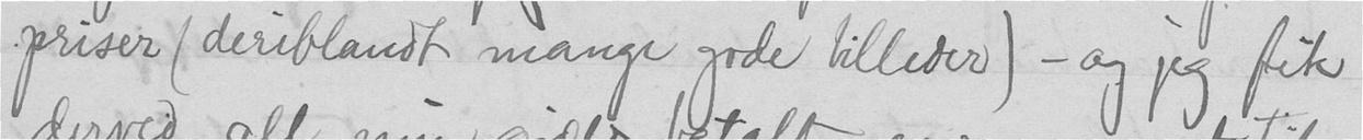priser (deriblandt mange gode billeder) - og jeg fik
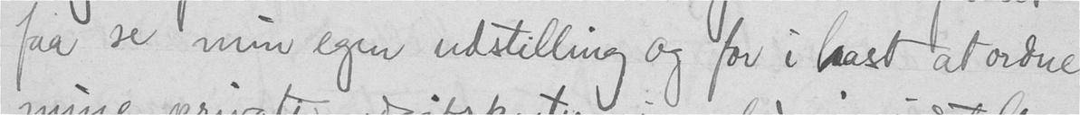faa se min egen udstilling og for i hast at ordne
